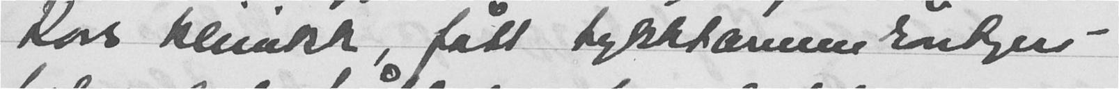Kors klinikk, fått tykktarmen røntgen-
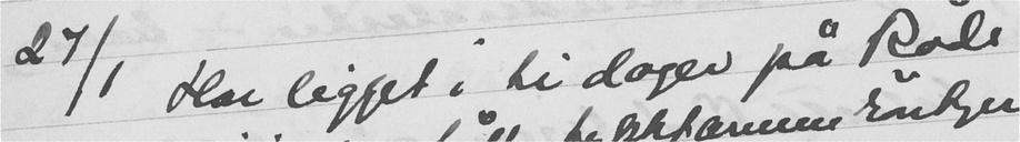27/1 Har ligget i ti dager på Røde
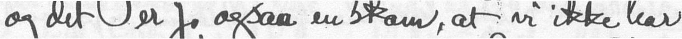og det er jo ogsaa en skam, at vi ikke har
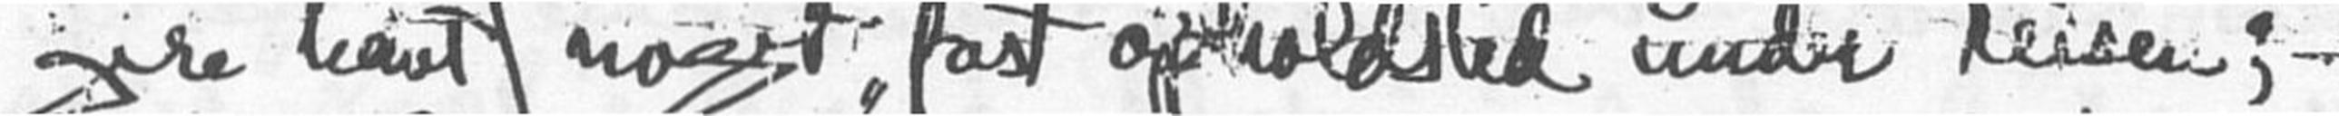gere havt noget "fast opholdssted" under reisen ;
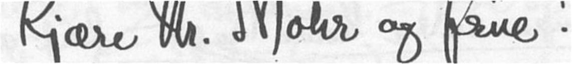Kjære Hr. Mohr og Frue !
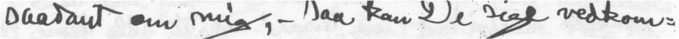saadant om mig, - saa kan De sige vedkom-
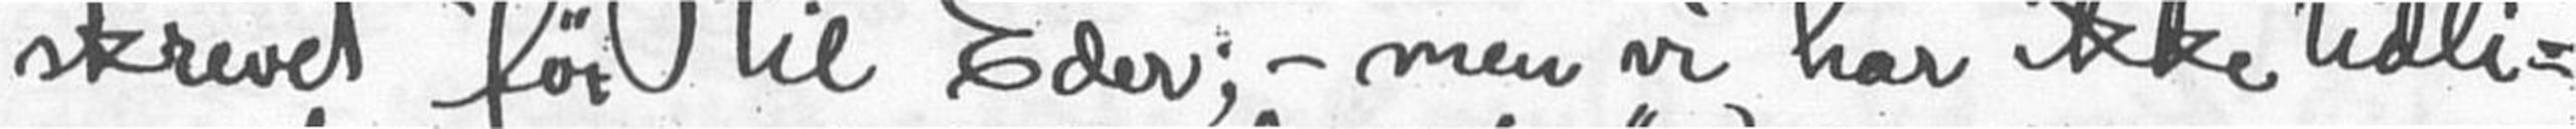skrevet før til Eder ; - men vi har ikke tidli-
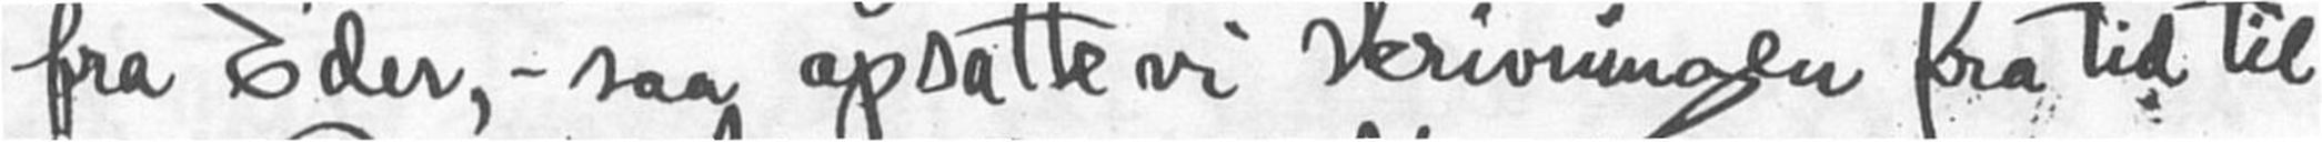fra Eder, - saa opsatte vi skrivningen fra tid til
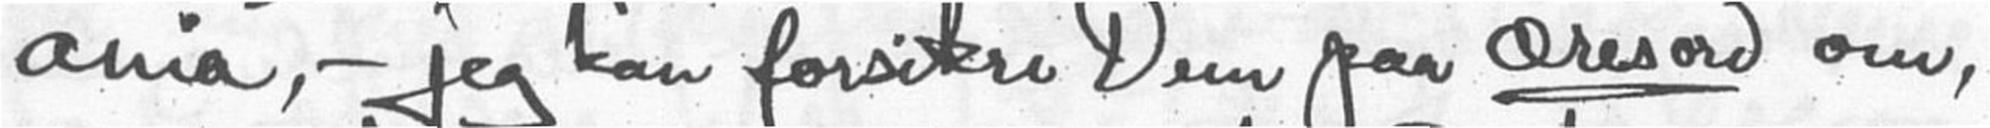ania, - jeg kan forsikre Dem paa æresord om,
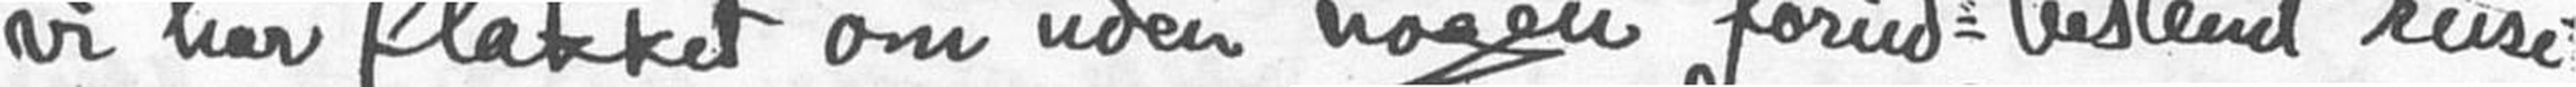vi har flakket om uden nogen forud-bestemt reise-
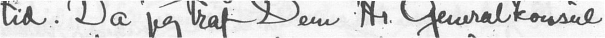tid. Da jeg traf Dem Hr. Generalkonsul
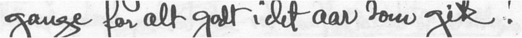gange for alt godt i det aar som gik ! -
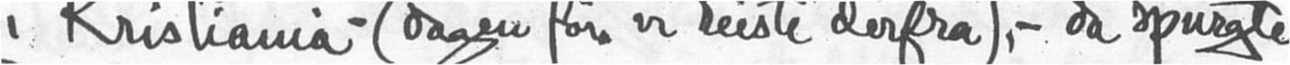i Kristiania - (dagen for vi reiste derfra), - da spurgte
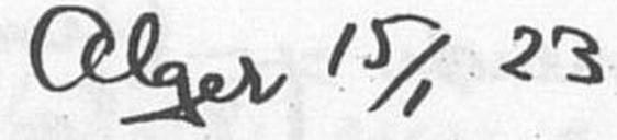Alger 15/1 23
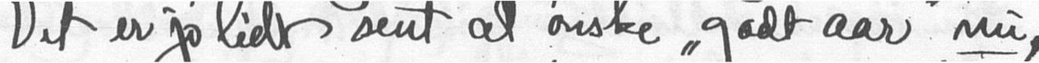Det er jo lidt sent at ønske "godt aar" nu,
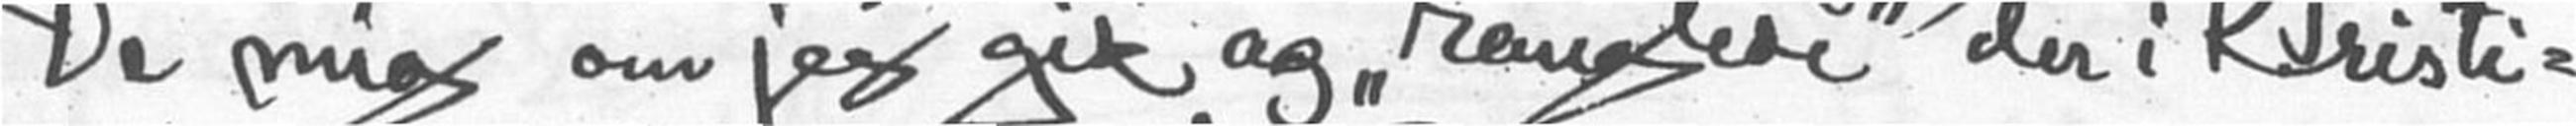De mig om jeg gik og "ranglede" der i Kristi-
